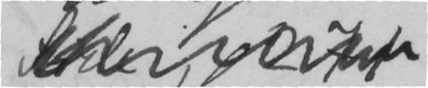dderser
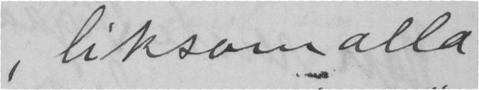, liksom alla
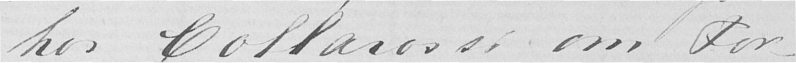hos Collarossi om For-
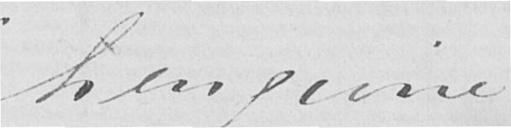hengivne
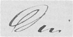Din
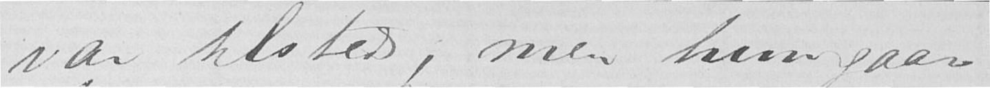var tilstede; men hun gaar
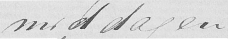middagen
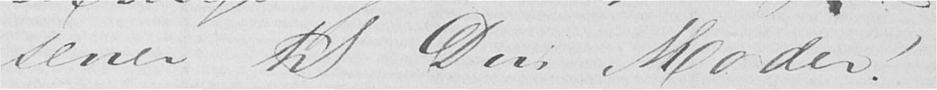sener til Din Moder!
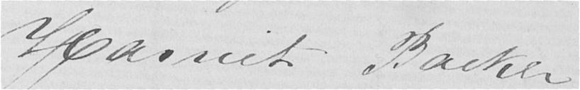Harriet Backer
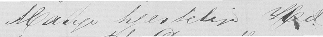Mange hjertelige Hil-
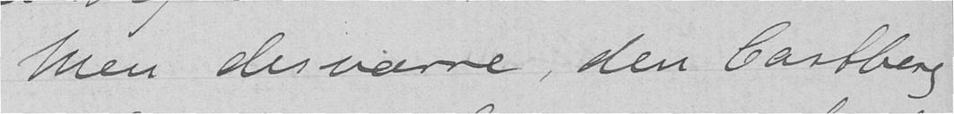Men desværre, den Castberg
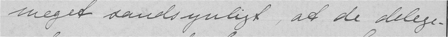meget sandsynligt, at de delege-
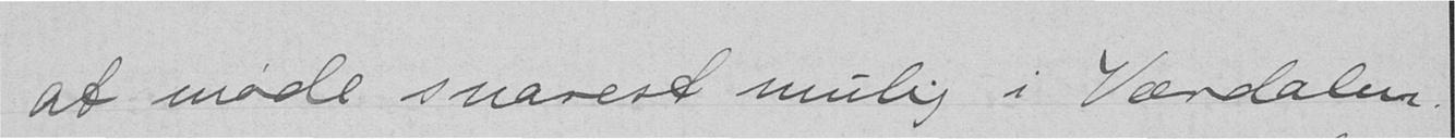at møde snarest mulig i Værdalen.
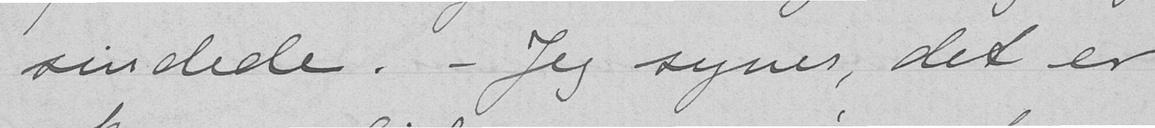sindede. - Jeg synes, det er
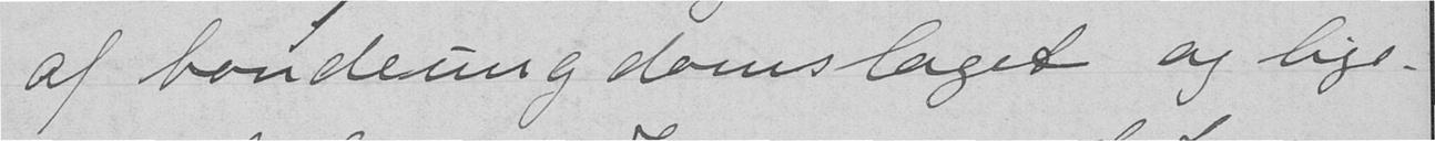af bondeungdomslaget og lige-
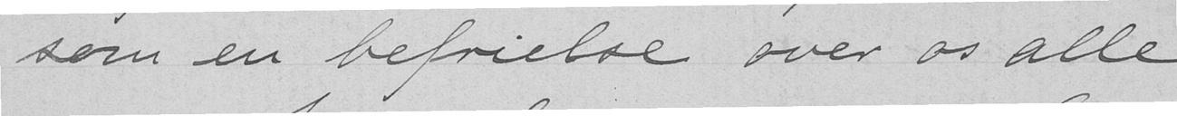som en befrielse over os alle.
